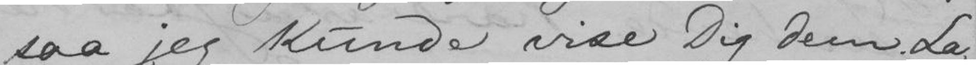saa jeg kunde vise Dig dem. La-
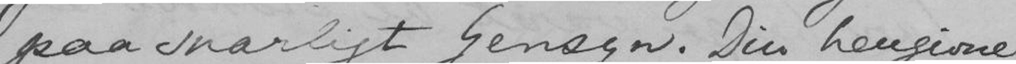paa snarligt Gensyn. Din hengivne
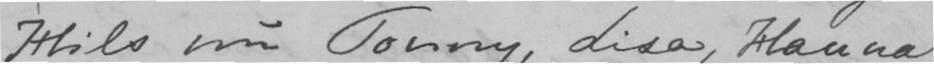Hils nu Tonny, Lisa, Hanna
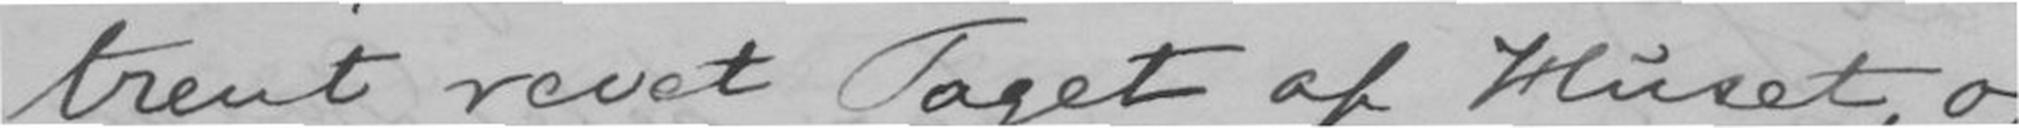trent revet Taget af Huset, og
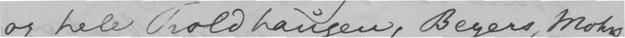og hele Troldhaugen, Beyers, Mohns
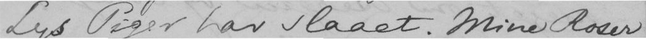Lys Piger har slaaet. Mine Roser
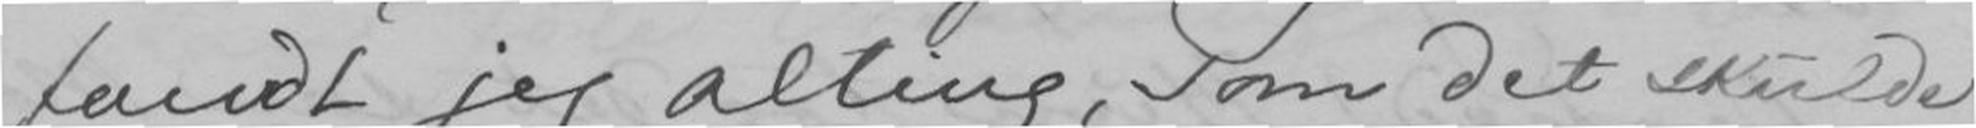fandt jeg alting, som det skulde
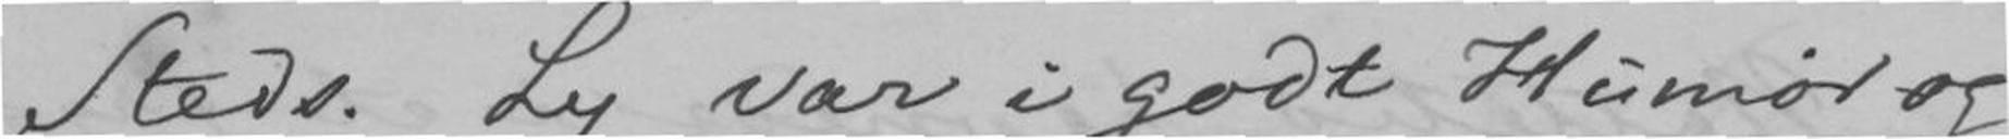Steds. Ly var i godt Humør og
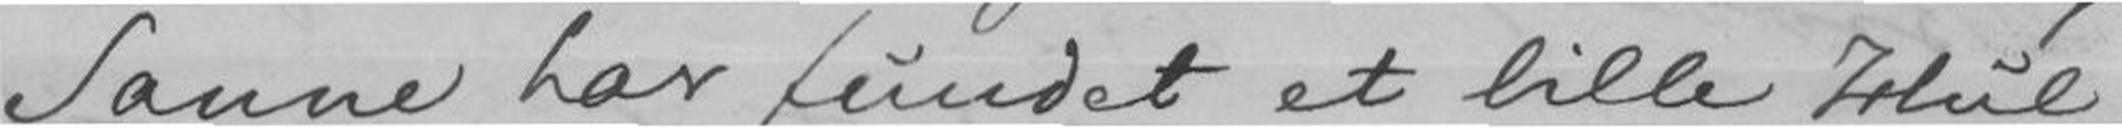Sanne har fundet et lille Hul
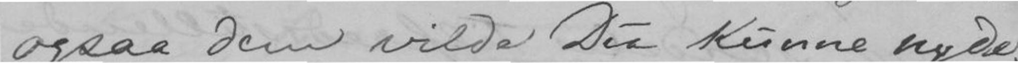ogsaa den vilde Du kunne nyde.
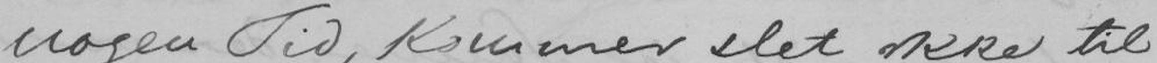nogen Tid, kommer slet ikke til
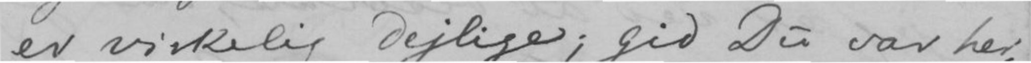er virkelig dejlige; gid Du var her,
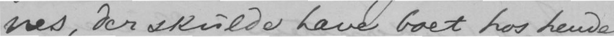nes, der skulde have boet hos hende
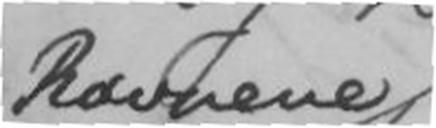Ravnene
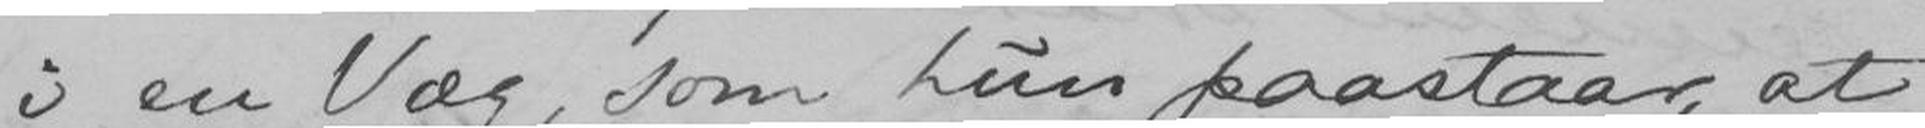i en Væg, som hun paastaar, at
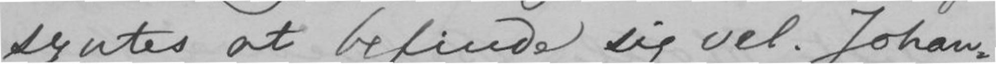syntes at befinde sig vel. Johan-
